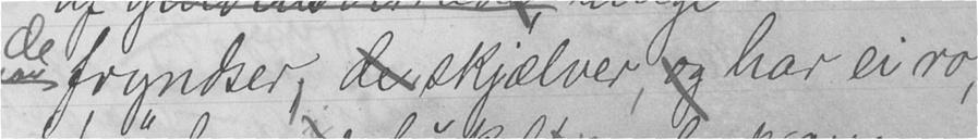de fryndser, skjælver, har ei ro,
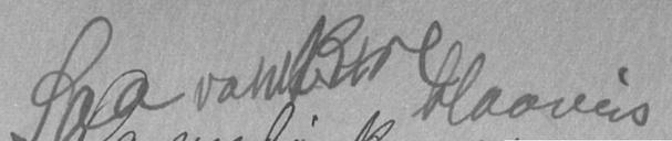Saa vakker blaaveis
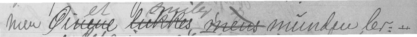Men øiet smiler, mens munden ler: -
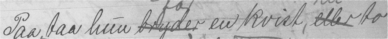Paa taa hun får en kvist, nei to
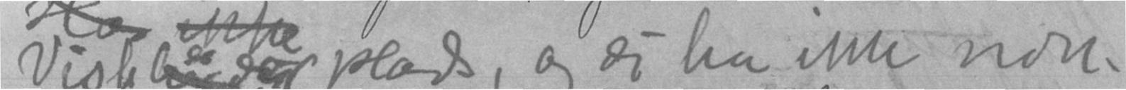Visk er der plads, og si ha ikke nok!
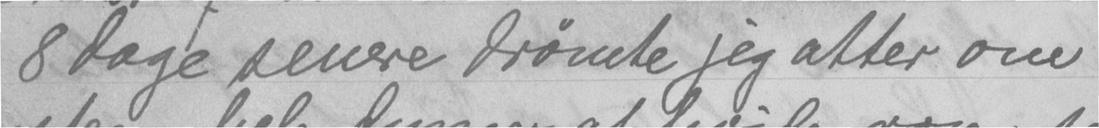8 dage senere drømte jeg atter om
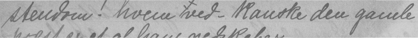stendom! hvem ved - kanske den gamle
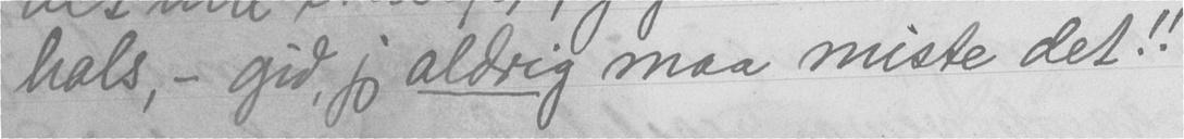hals, - gid jeg aldrig maa miste det!!
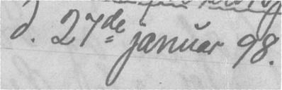d. 27de januar 98.
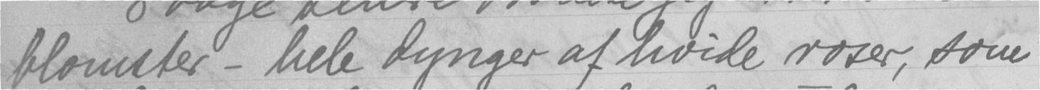blomster - hele dynger af hvide roser, som
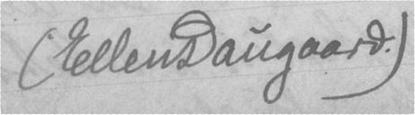(Ellen Daugaard.)
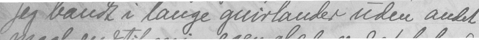jeg bandt i lange guirlander uden andet
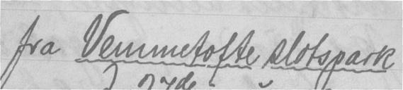fra Vemmetofte slotspark.
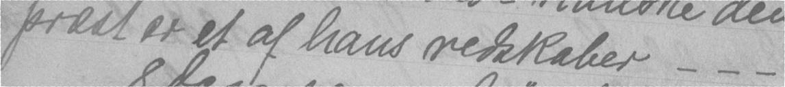præst er et af hans redskaber - - -
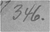346.
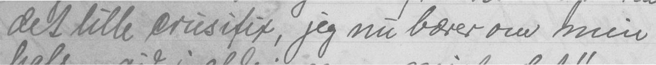det lille crusifix, jeg nu bærer om min
